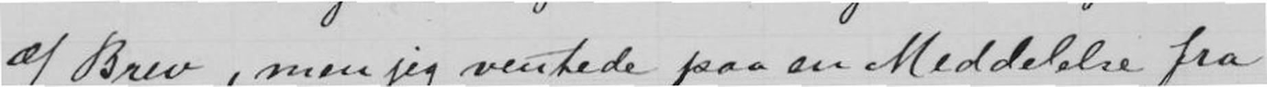d/ Brev, men jeg ventede paa en Meddelelse fra
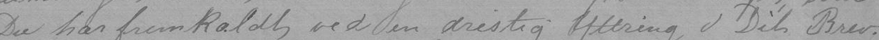Du har fremkaldt ved en dristig Yttring i Dit Brev.
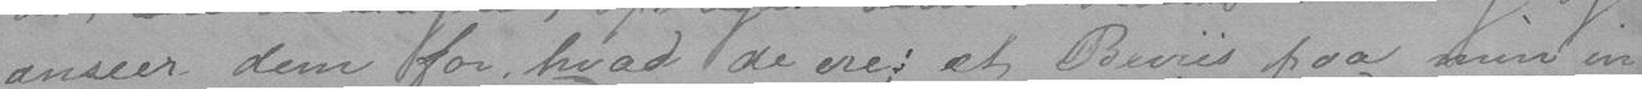anseer dem for hvad de ere et Bevis paa min in-
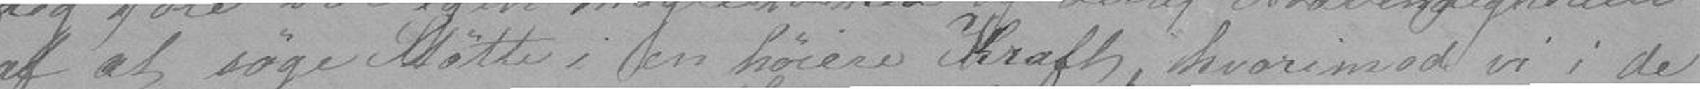af at søge støtte i en høiere Kraft, hvormed vi i de
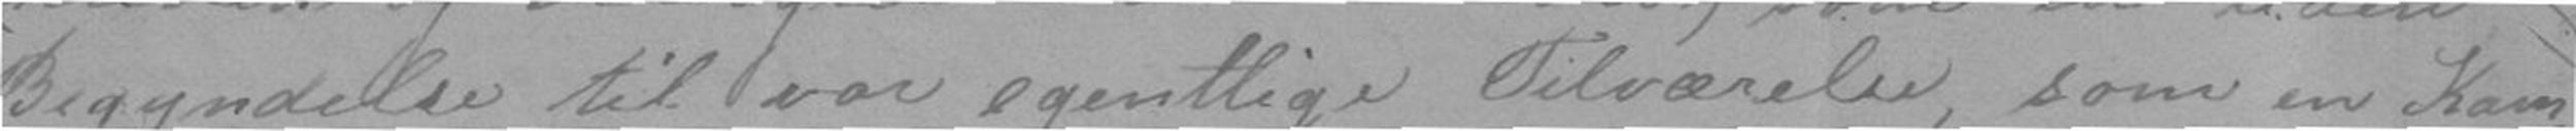Begyndelse til vor egentlige Tilværelse, som en Kam-
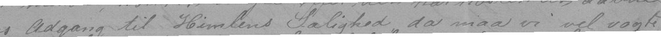Adgang til Himlens Salighed, da maa vi vel vogte
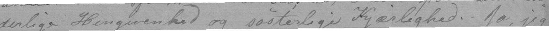derlige Hengivenhed og søsterlige Kjærlighed. Ja, jeg
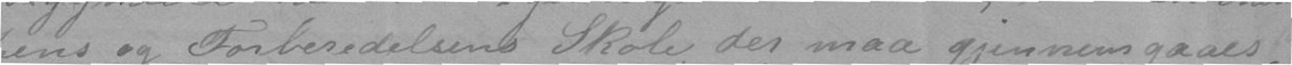pens Forberedelsens Skole, der maa gjennemgaaes,
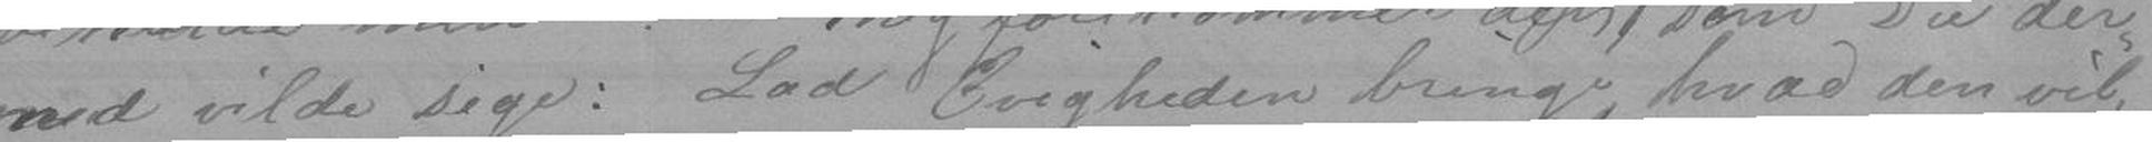med vilde sige: Lad Evigheden bringe hvad den vil,
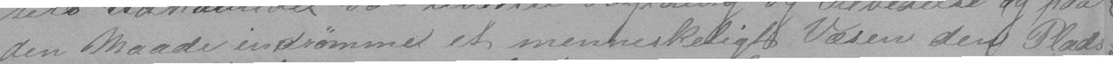den Maade indrømme et menneskeligt Væsen den Plads
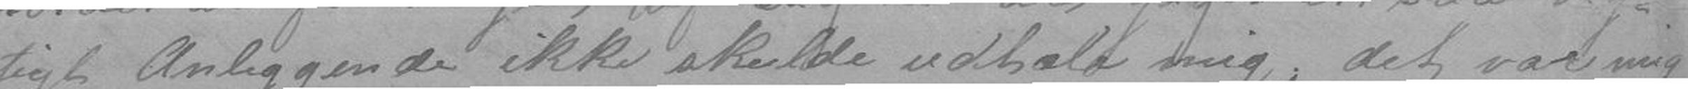tigt Anliggende ikke skulde udtale mig; det var mig
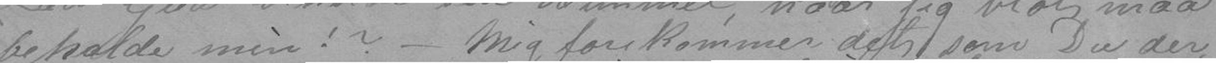beholde min!? - Mig forekommer det som Du der-
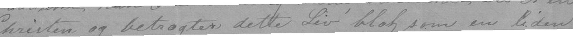Christen og betragter dette Liv blot som en liden
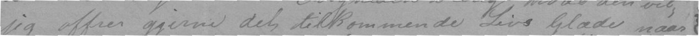jeg offrer gjerne det tilkommende Livs Glæde naar
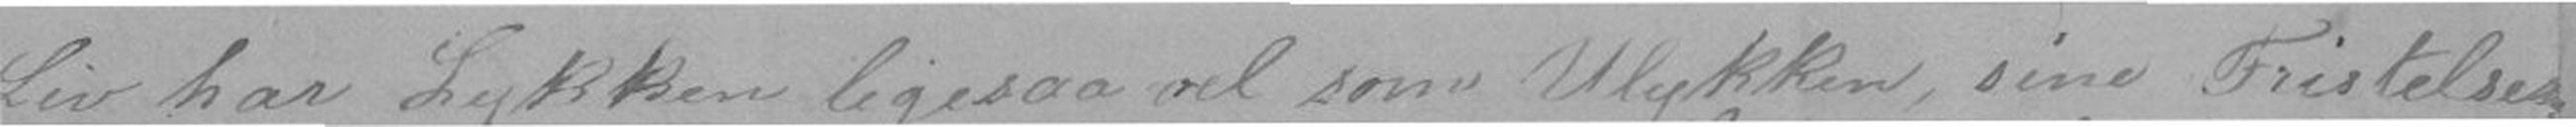Liv har Lykken ligesaa vel som Ulykken, sine Fristelser
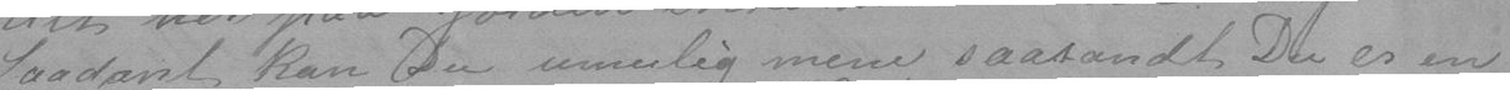Saadant kan Du umulig mene saasandt Du er en
